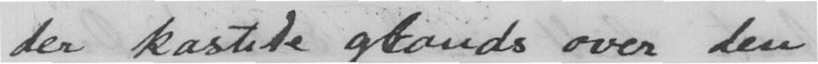der kastede glands over den
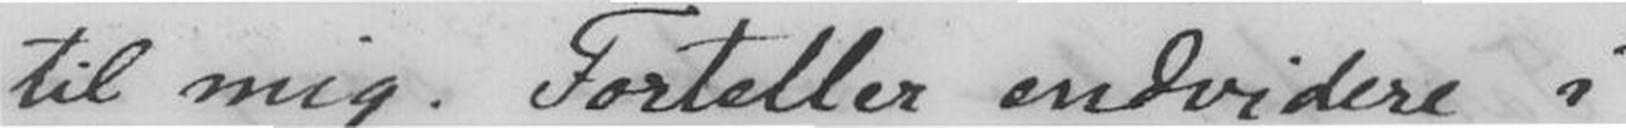til mig. Forteller endvidere i
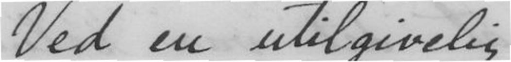Ved en utilgivelig
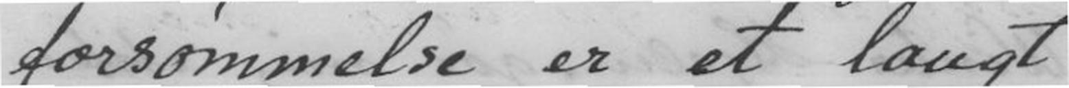forsømmelse er et langt
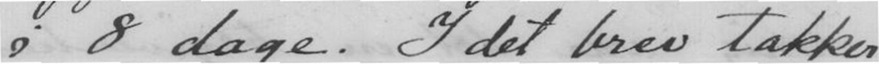i 8 dage. I det brev takker
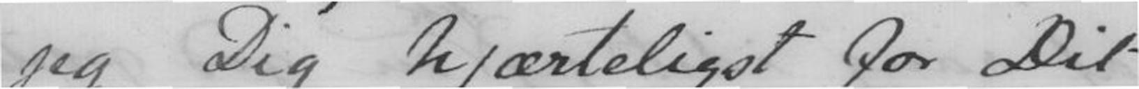jeg Dig hjærteligst for Dit
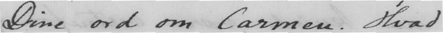Dine ord om Carmen. Hvad
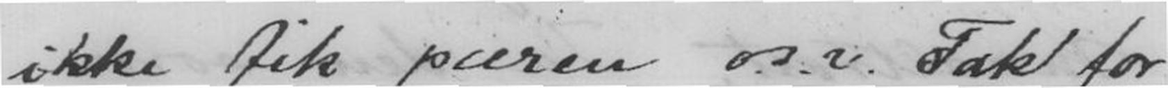ikke fik pæren o.s.v. Tak for
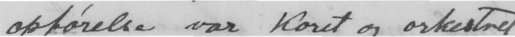opførelse var koret og orkestret
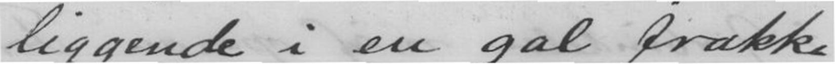liggende i en gal frakke
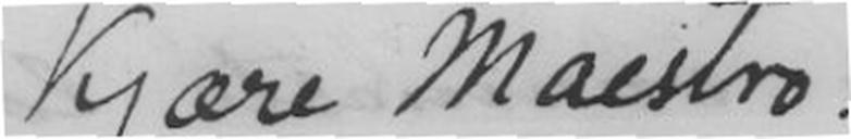Kjære Mastro
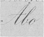Åbo
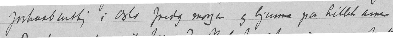forhaabentlig i Oslo fredag morgen og hjemme paa Lillehammer
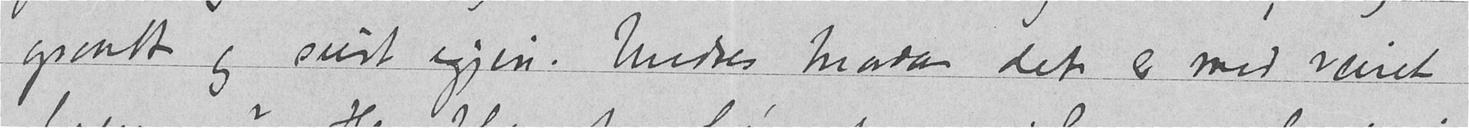graatt og surt igjen. Undres hvordan det er med veiret
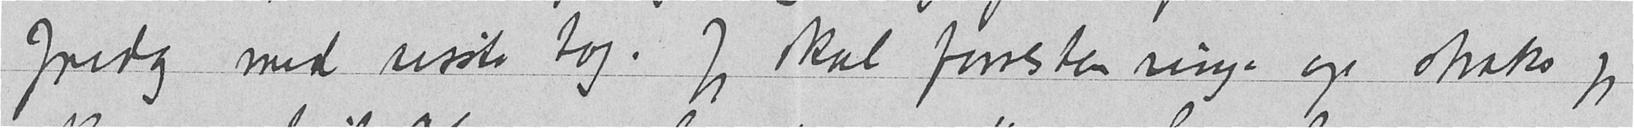fredag med sisste tog.  Jeg skal forresten ringe op straks jeg
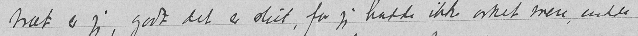træt er jeg, godt det er slut, for jeg hadde ikke orket mere, endda
